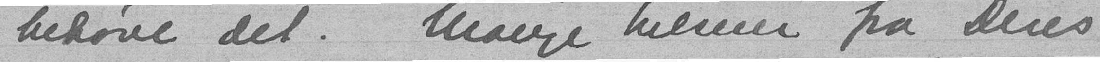behøve det. Mange hilsner fra Deres
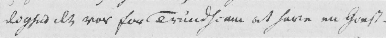dighed det var for Trundhiem at have en Giest-
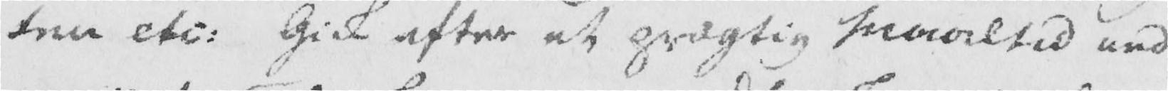ten etc. Gik efter et prægtig Maaltid ned
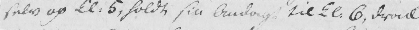selv op Kl. 5, holdt sin Andagt til Kl. 6, drack
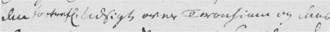den fortrefl. udsigt over Tronhiem og dens
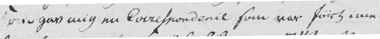Han gav mig en Correspondence som var ført ime-
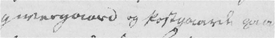givergaard og Postgaard paa
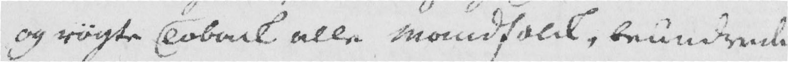og røgte Tobak alle Mandfolk, beundrede
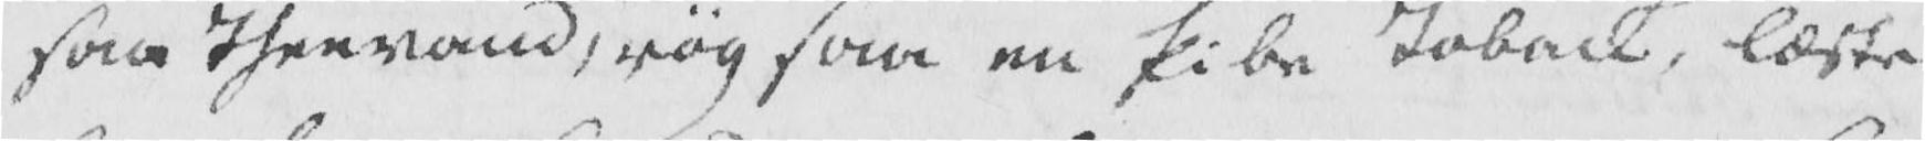saa Theevand, røg saa en Pibe Toback, læste
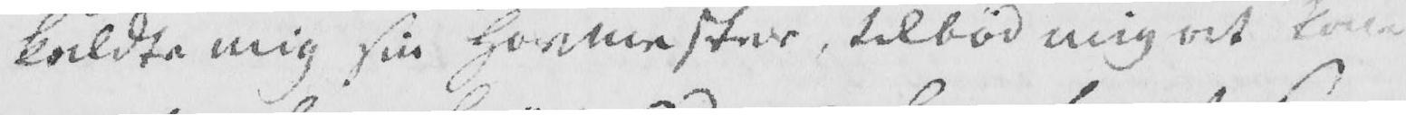kaldte mig sin Hovmester, tilbød mig at kom-
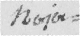Naja-
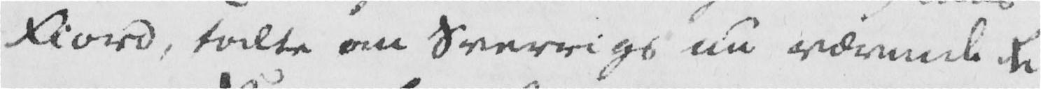Fiord, talte om Sverrigs nu værende Re-
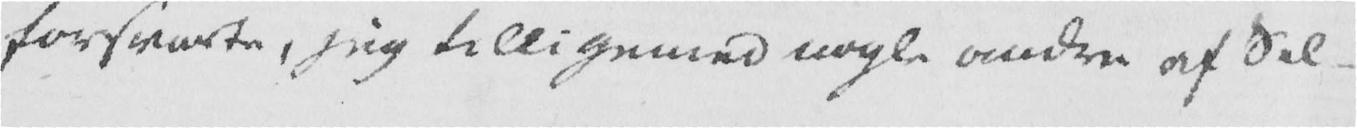forsvarte, jeg tilligemed nogle andre af Sel-
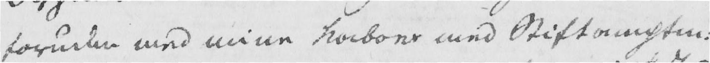foruden med mine naboer med Stiftamptm.
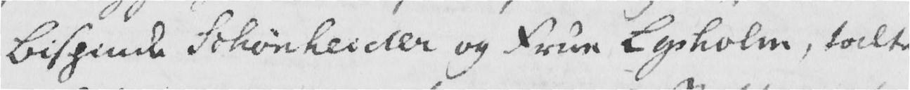bispinde Schønheider og Frue Lysholm, talte
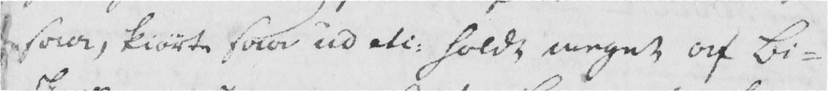Aviser, kiørte saa ud etc. holdt meget af Bi-
